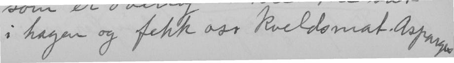i hagen og fekk oss kveldsmat. Asparges
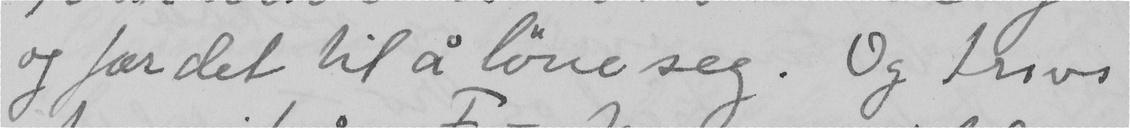og fær det til å löne seg. Og trivs
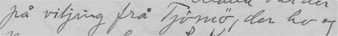på vitjing frå Tjömö; der ho og
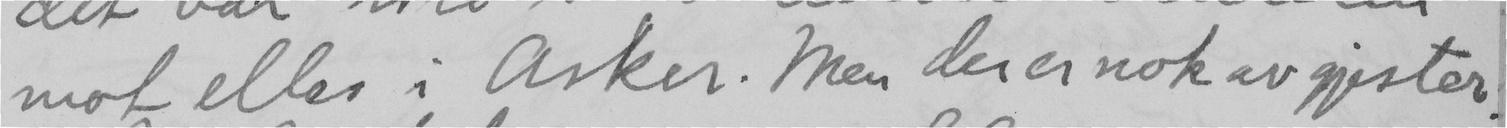mot elles i Asker. Men dei er nok av gjester.
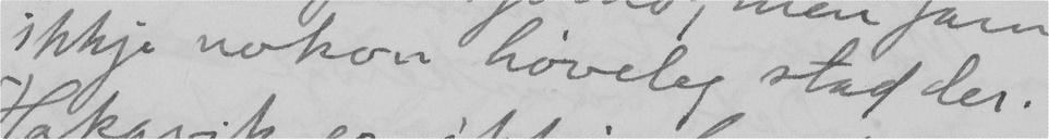ikkje nokon höveleg stad der.
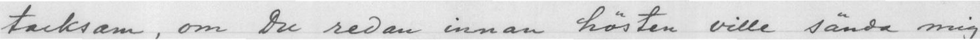tacksam, om Du redan innan hösten ville sända mig
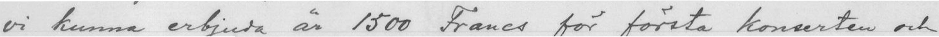vi kunna erbjuda är 1500 Francs för första konserten och
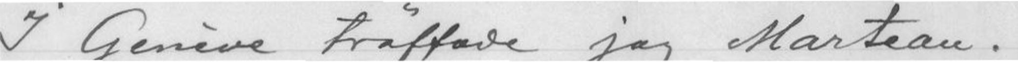I Geneve träffake jag Marteau.
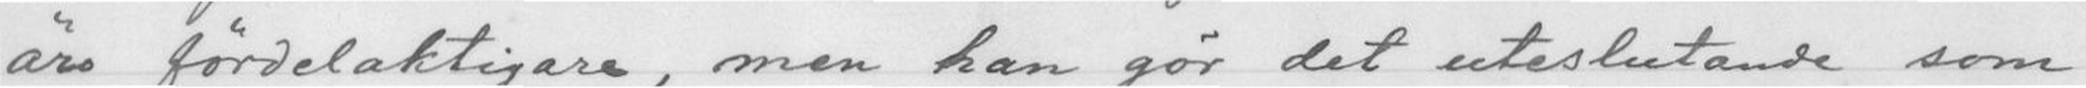ärs fördelaktigare, men han gör det uteslutande som
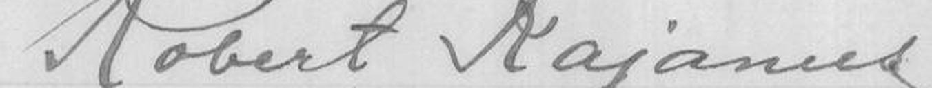Robert Kajanus
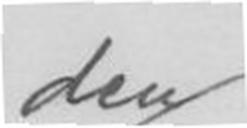den
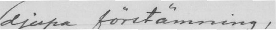djupa förstämning,
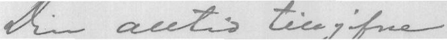Din alltid tillgifne
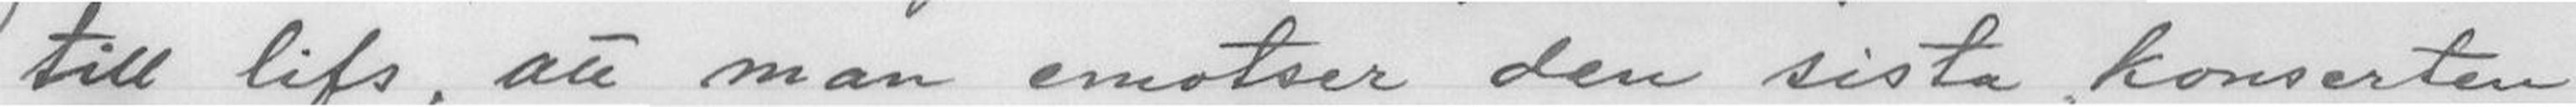till lifs, att man emotser den sista konserten
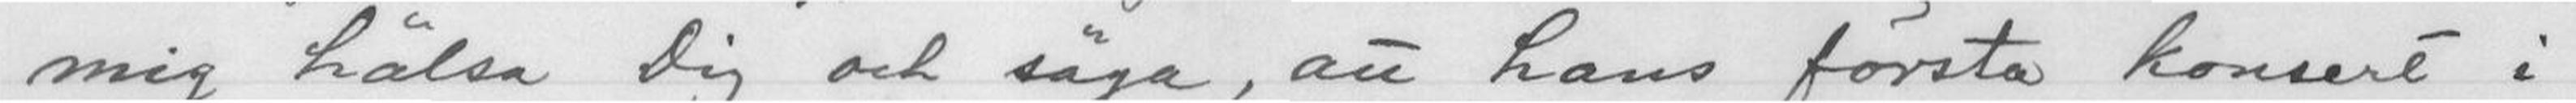mig hälse Dig och säga, att hans första komsert i
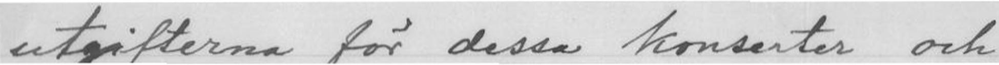utgifterna för dessa konserter och
